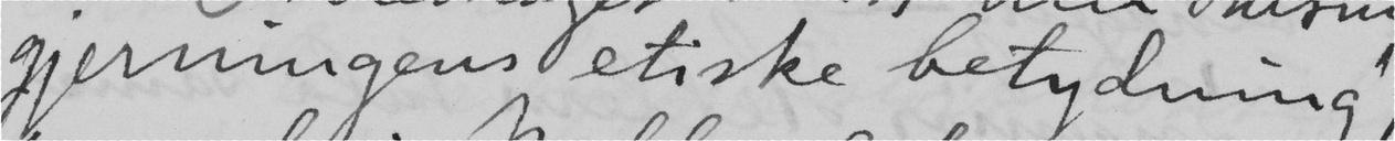gjerningens etiske betydning",
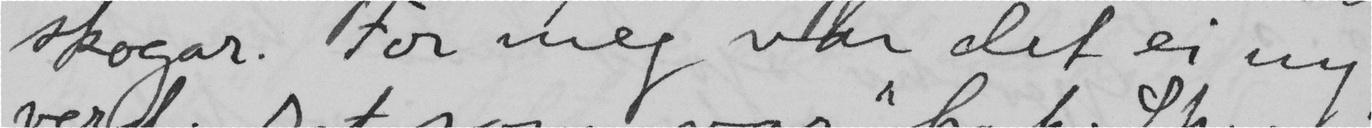skogar. For meg var det ei ny
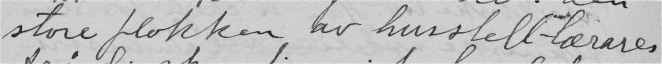store flokken av husstell-lærares
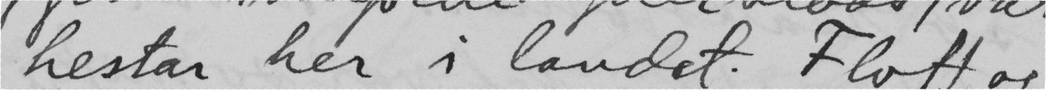hestar her i landet. Flott og
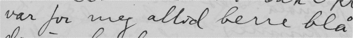var for meg altid berre blå
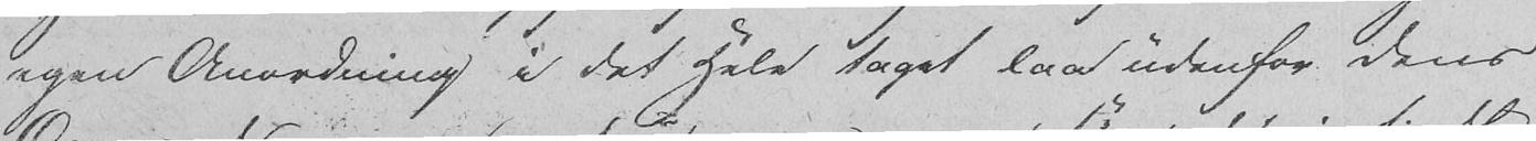egen Anordning i det Hele taget laa udenfor dens
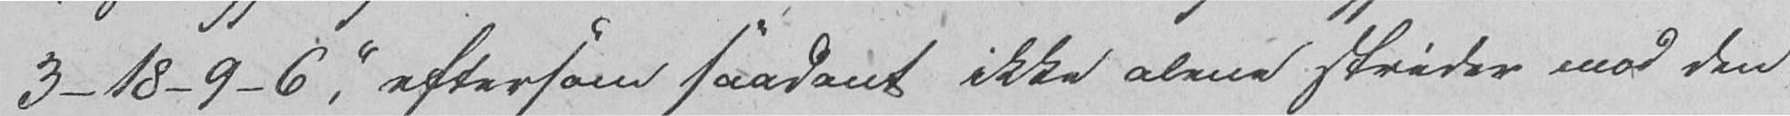3-18-9-6, "eftersom saadant ikke alene strider mod den
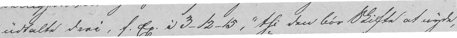udtalte deri, f. Ex. i 3-12-15, "thi den bør Skifte at nyde,
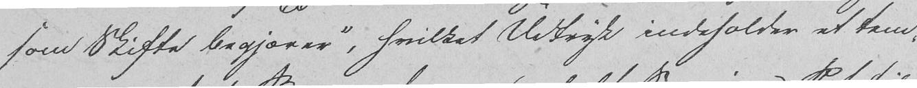som Skifte begjærer", hvilket Udtryk indeholder et tem-
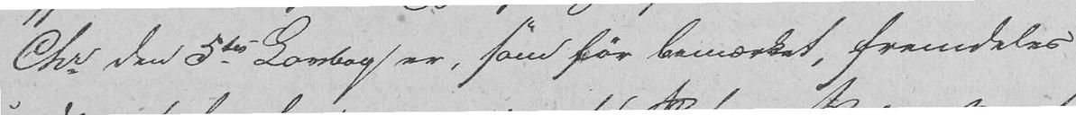Chr den 5tes Lovbog er, som før bemærket, fremdeles
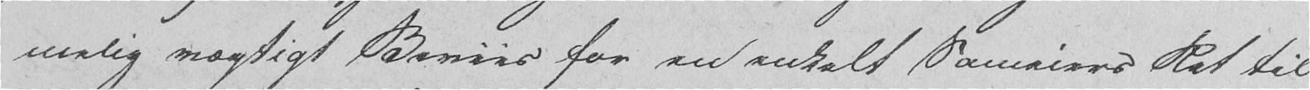melig vægtigt Beviis for en enkelt Sameiers Ret til
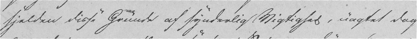sjelden disse Grunde af synderlig Vigtighed, uagtet dog
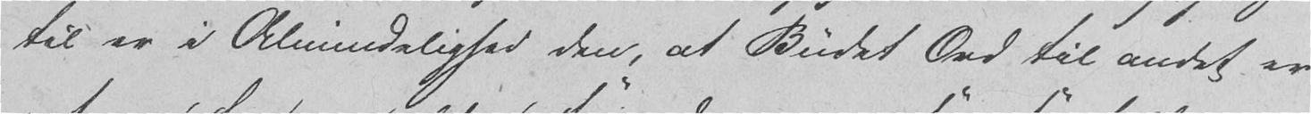til er i Almindelighed den, at Budet Ord til andet er
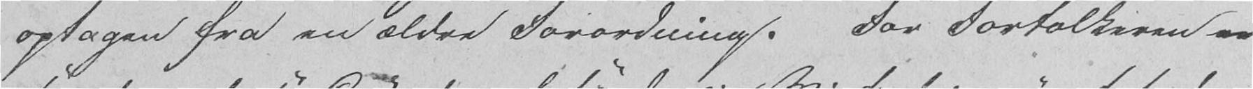optagen fra en ældre Forordning. For Fortolkeren er
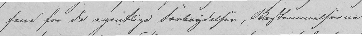fene for de egentlige Forbrydelser, Bestemmelserne
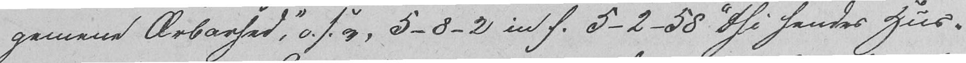gemene Ærbarhed", o. s. v. 5-8-2 in f. 5-2-58 "thi hendes Hus-
